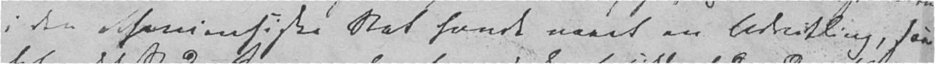i den atheniensiske Stat havde naaet en Udvikling, som
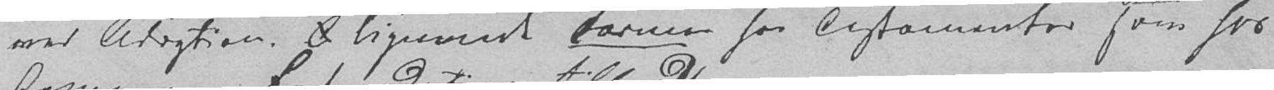ved Adoption. I Lignende Former for Testamenter som hos
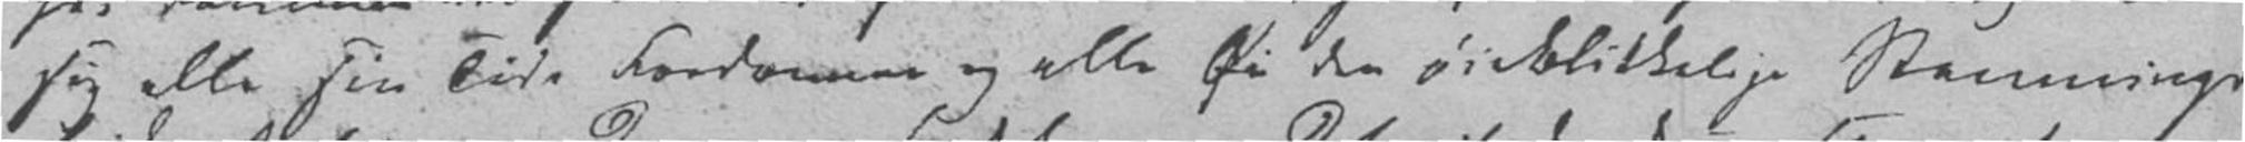sig alle sin Tids Fordommer og alle den øieblikkelige Stemnings
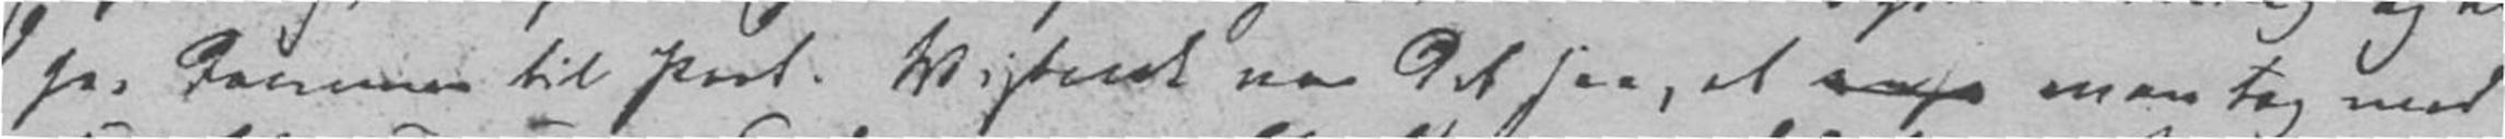fra Dommer til Prest. Vistok var det saa, at man tog med
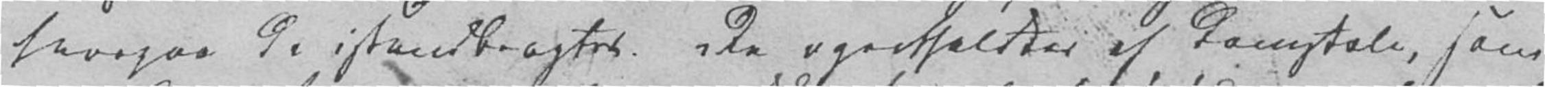hvorpaa de istandbragtes. De opretholdtes af Domstole, som
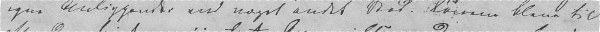egne Anliggender end noget andet Sted. Lovene bleve til
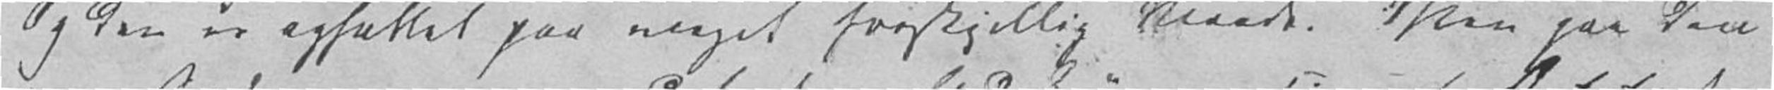Og den er opfattet paa meget forskjellig Maade. Men paa den
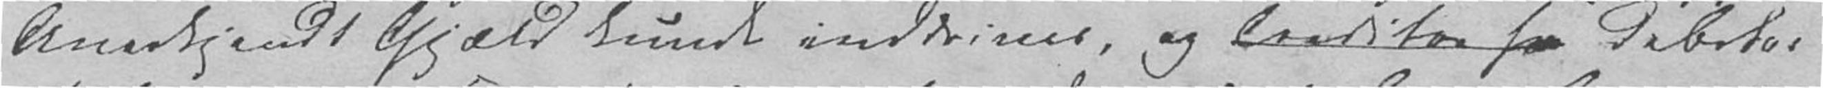Anerkjendt Gjæld kunde inddrives, og Debitor
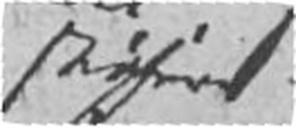større
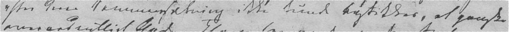efter deres Sammensætning ikke kunde bestikkes, et ganske
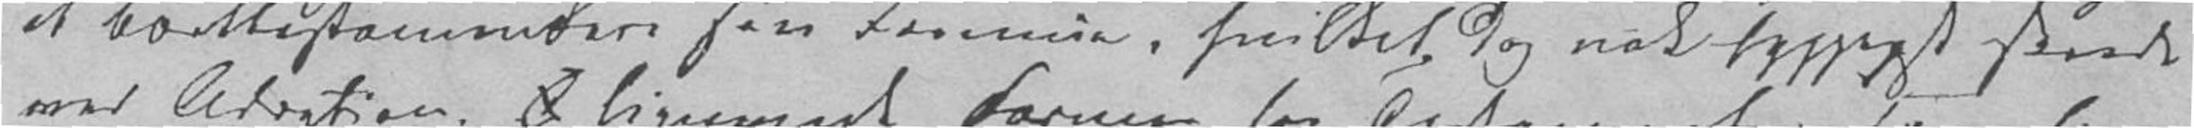at borttestamentere fin Formue, hvilket dog nok hyppigst skeede
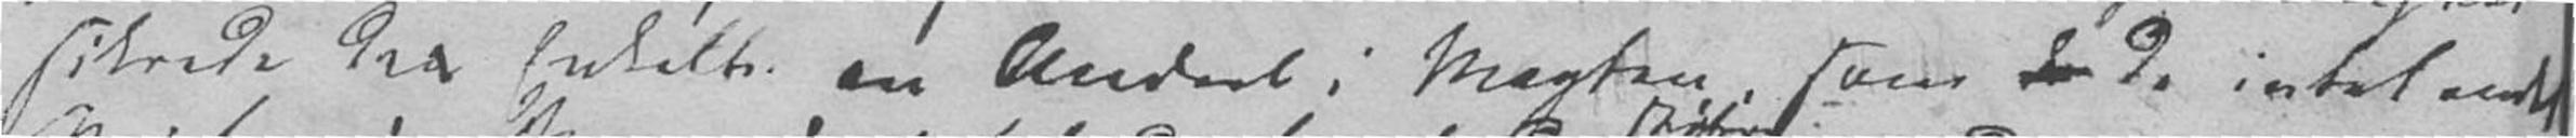sikrede den Enkelte en Andeel i Magten, som de intet andet
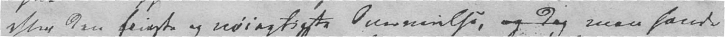efter den frieste og nøiagtigste Overveielse, man havde
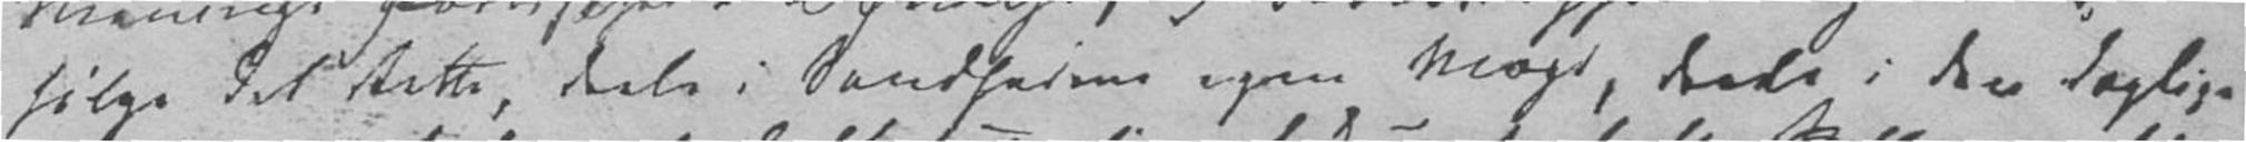følge det Rette, deels i Sandhedens egen Magt, deels i den daglige
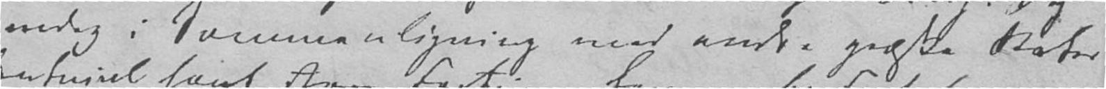endog i Sammenligning med andre græske Stater
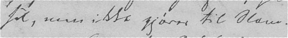sel, men ikke gjøres til Slave.
